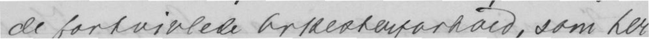de fortvivlede orkesterforhold, som her
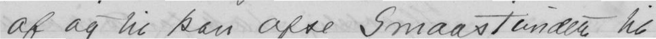af og til ofre Smaastunden til
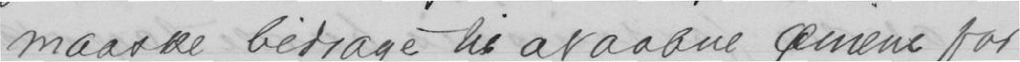maaske bidrage til at aabne øinene for
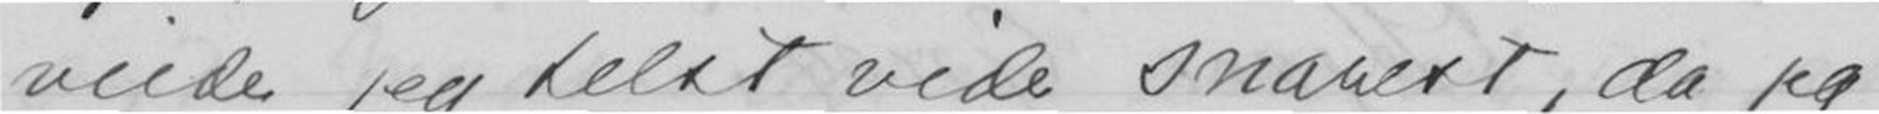vilde jeg helst vide snarest, da jeg
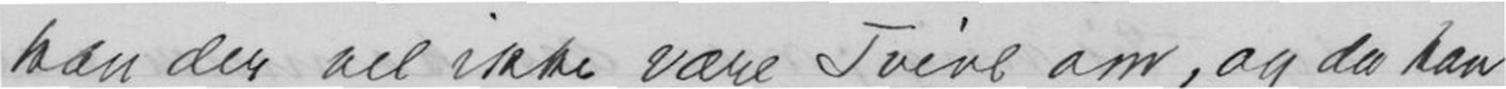kan det vel ikke være Tvivl om, og da kan
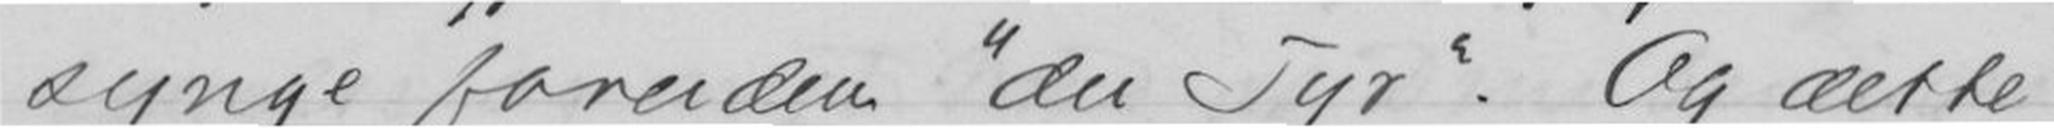synge foruden "du Tyr". Og dette
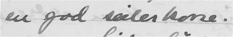en god seilerkone.
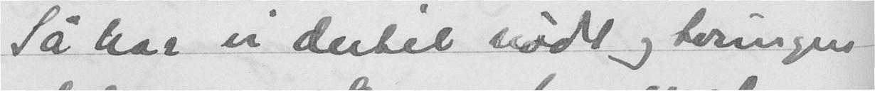Så har vi dertil nødt og teringen
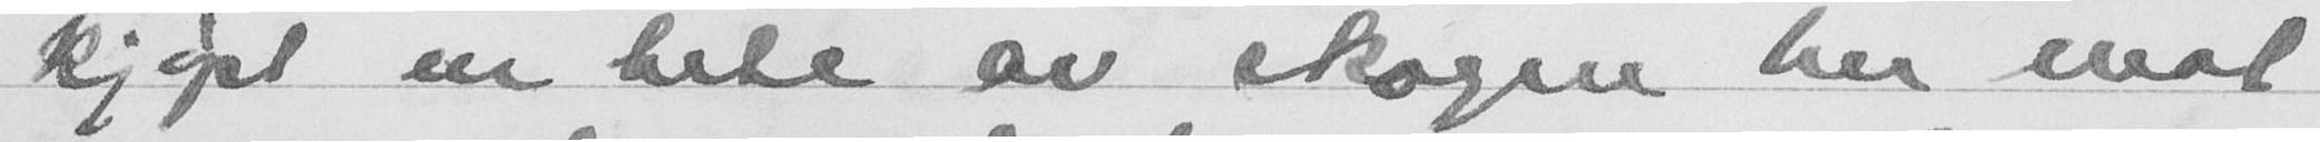kjøpt en bete ev skogen her mot
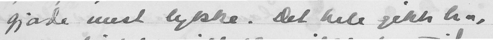gjorde mest lykke. Det hele gikk bra,
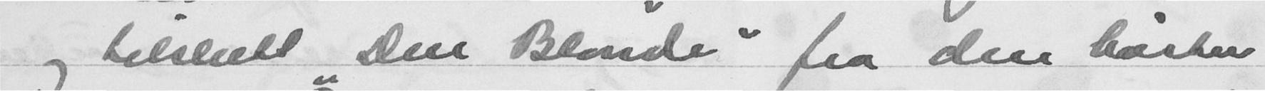og tilslutt "Den Blonde" fra den høsten
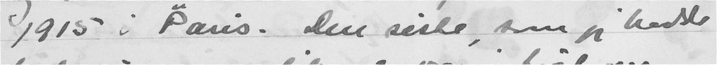1915 i Paris. Den siste, som jeg hadde
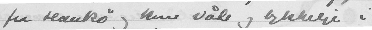fra Hankø og kom våte og lykkelige i
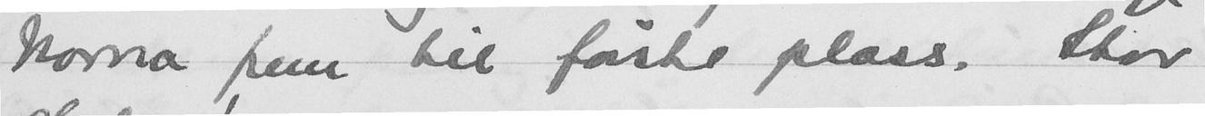Norna frem til første plass. Stor
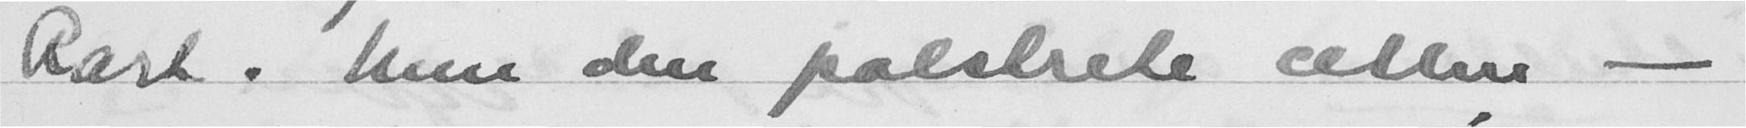Rart. Men den polstrete cellen -
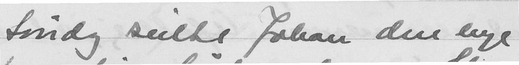Søndag seilte Johan den nye
